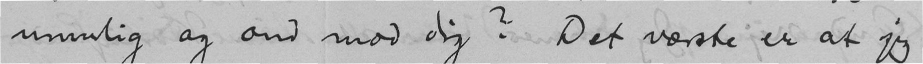umulig og ond mod dig? Det værste er at jeg
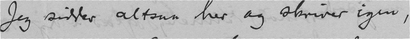Jeg sidder altsaa her og skriver igen,
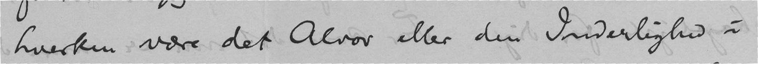hverken være det Alvor eller den Inderlighed i
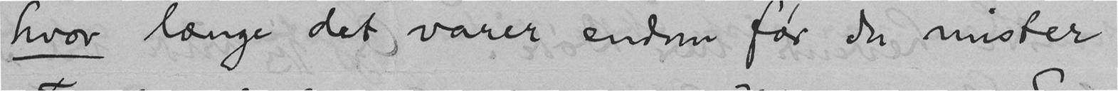hvor længe det varer endnu før du mister
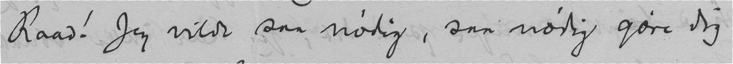Raad! Jeg vilde saa nødig, saa nødig gøre dig
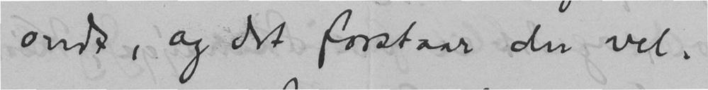ondt, og det forstaar du vel.
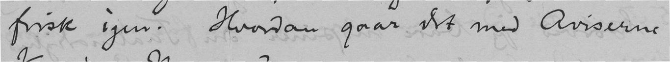frisk igen. Hvordan gaar det med Aviserne
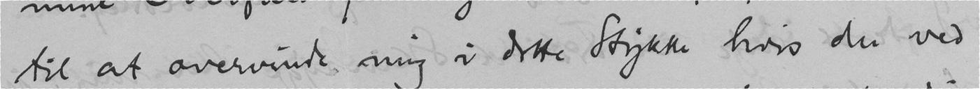til at overvinde mig i dette Stykke hvis du ved
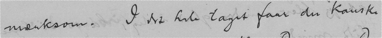mærksom. I det hele taget faar du kanske
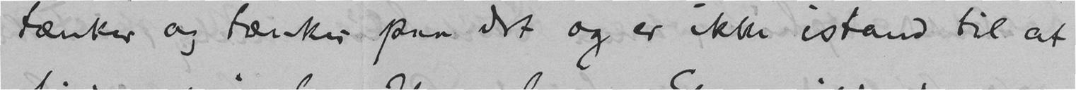tænker og tænker paa det og er ikke istand til at
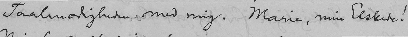Taalmodigheden med mig. Marie, min Elskede!
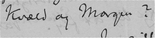Kvæld og Morgen?
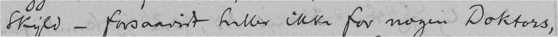Skyld - forsaavidt heller ikke for nogen Doktors,
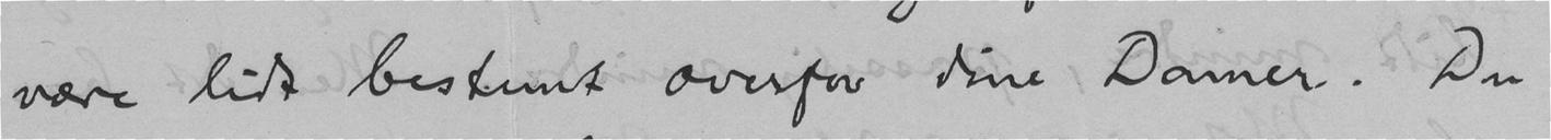være lidt bestemt overfor dine Damer. Du
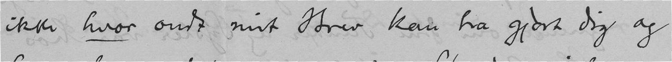ikke hvor ondt mit Brev kan ha gjort dig og
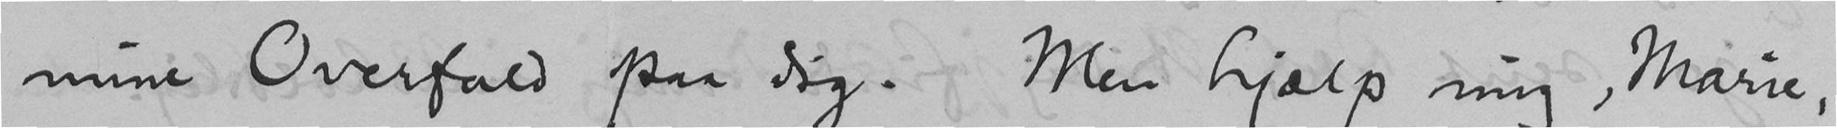mine Overfald paa dig. Men hjælp mig, Marie,
